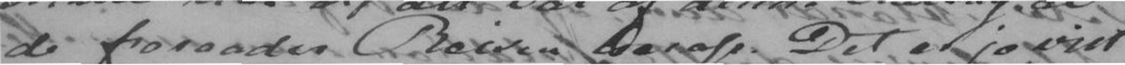de fraraades Reisen herop. Det er jo vist
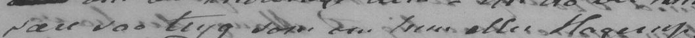være saa tryg som om hun eller Hagerup
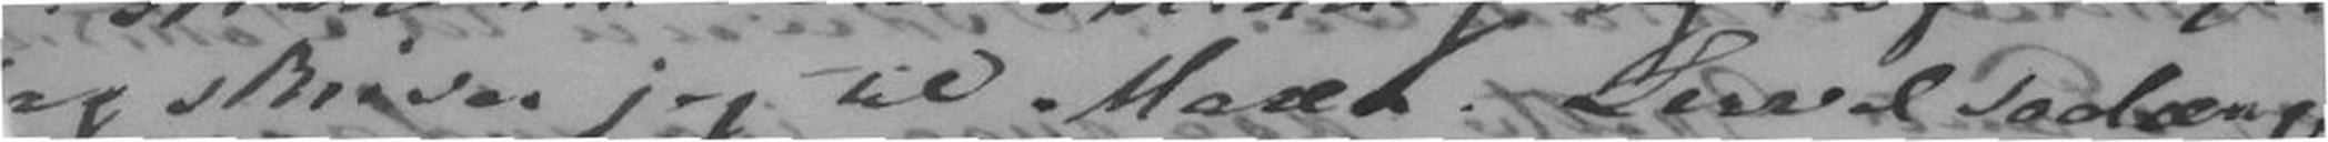ag skriver jeg til Maren. Levvel saalænge
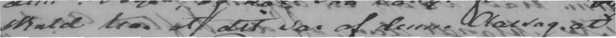skulde troe at det var af denne Aarsag at
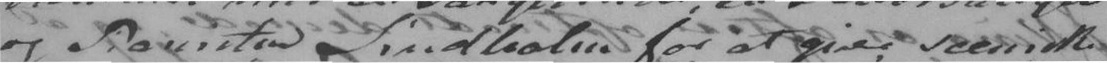og Pianisten Lindholm for at give sceniske
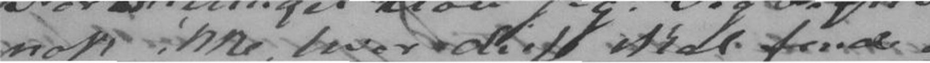nok ikke hvor disse skal finde
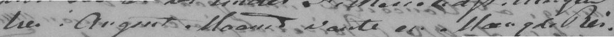her i August Maaned vente en Mængde Rei-
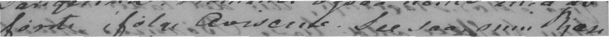første ifølge Aviserne. See saa min kjære
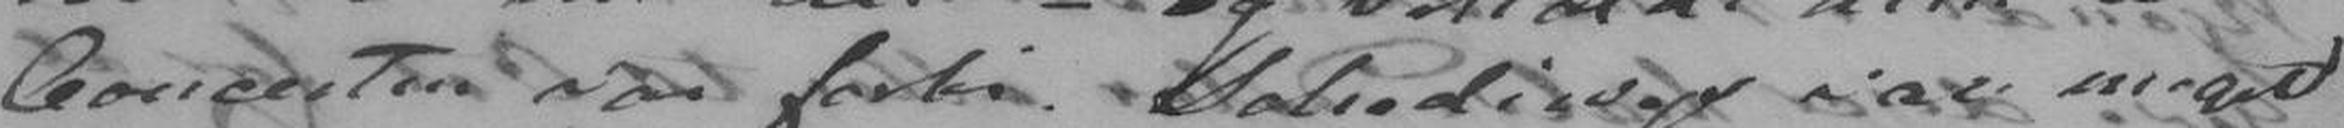Concerten var forbi. Schediwys var meget
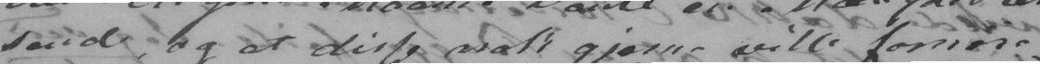sende, og at disse nok gjerne ville fornøie
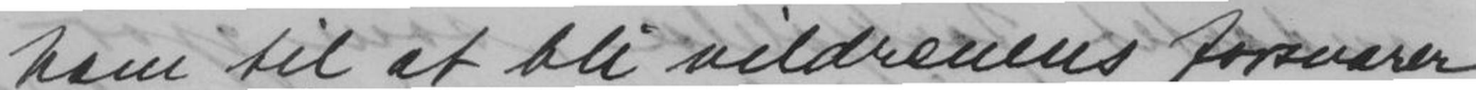ham til at bli vildrenens forsvarer
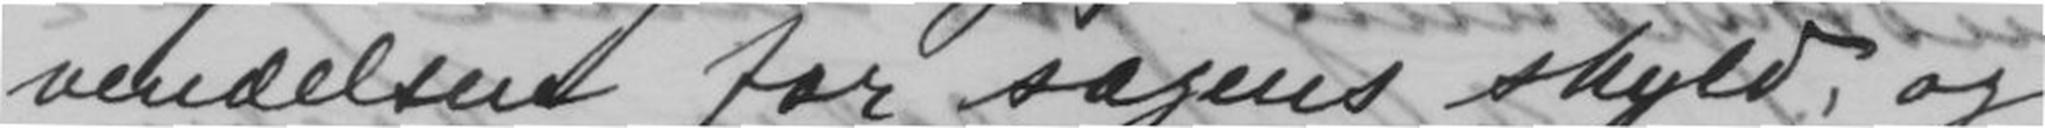vendelsene for sagens skyld, og
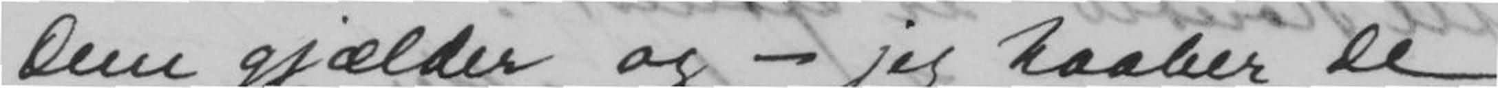Dem gjælder og - jeg haaber De
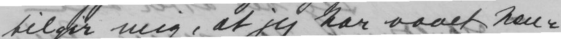tilgir mig, at jeg har vovet hen-
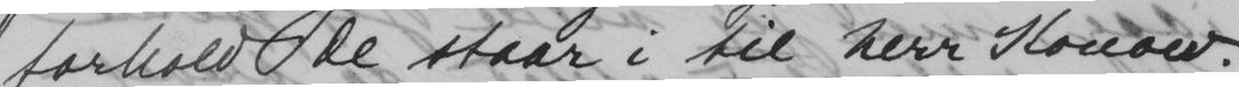forhold De staar i til herr Konow.
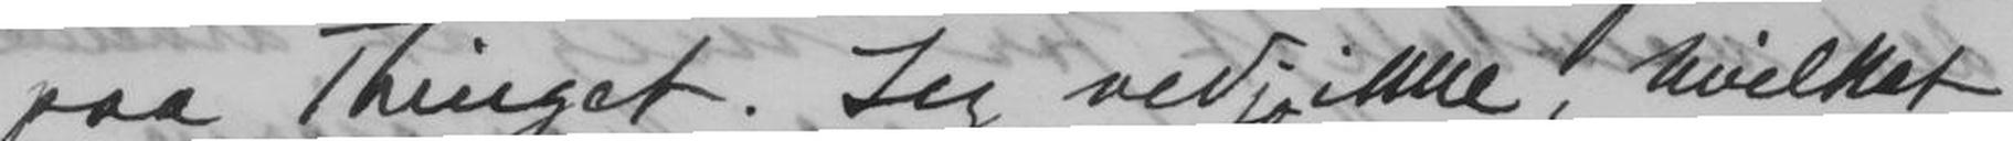paa Thinget. Jeg ved jo ikke, hvilket
